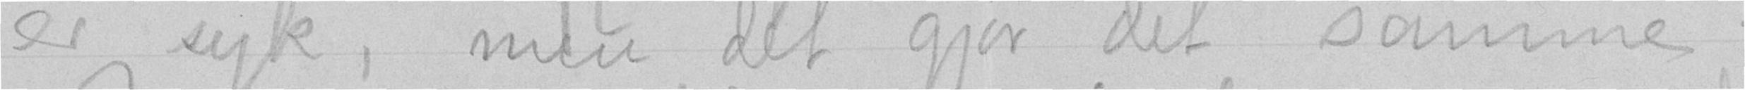er syk, men det gjør det samme.
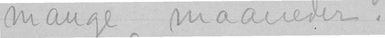mange maaneder.
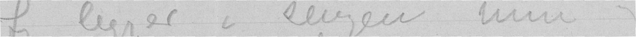Jeg ligger i sengen min og
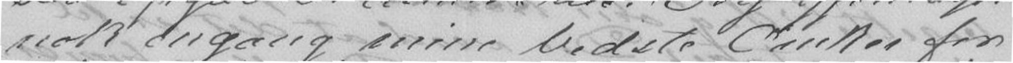nok engang mine bedste Ønsker for
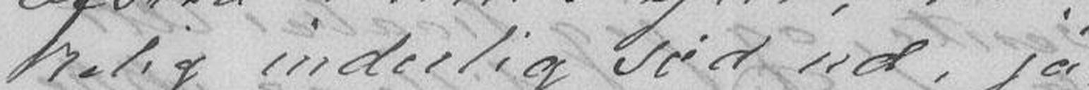kelig inderlig sød ud, ja
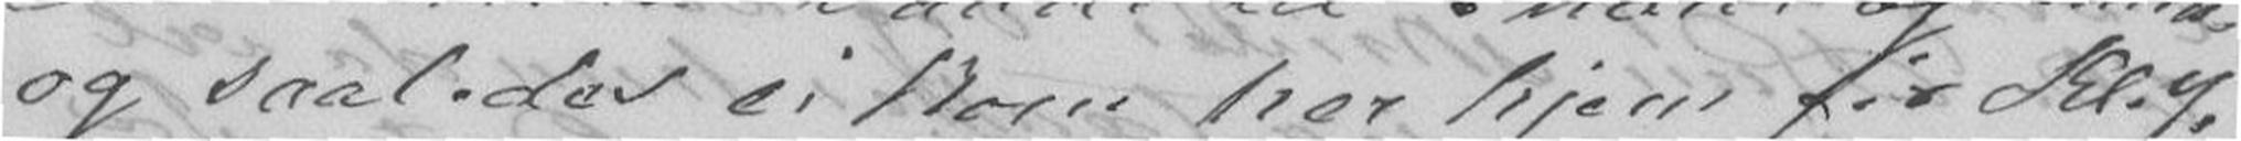og saaledes ei kom her hjem før Kl.7.
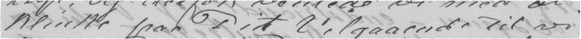klinke paa Dit Velgaaende til vi
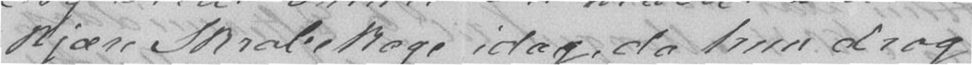kjære Skrabekage idag, da hun drog
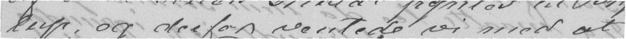lup, og derfor ventede vi med at
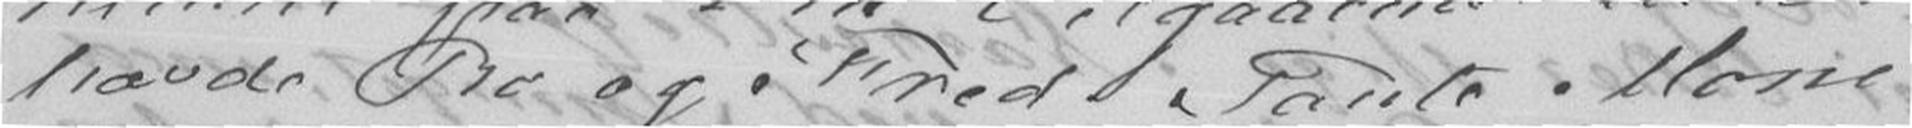havde Ro og Fred. Tante Mone
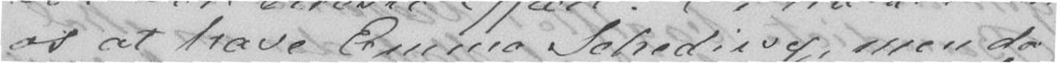os at have Emma Schediwy, men da
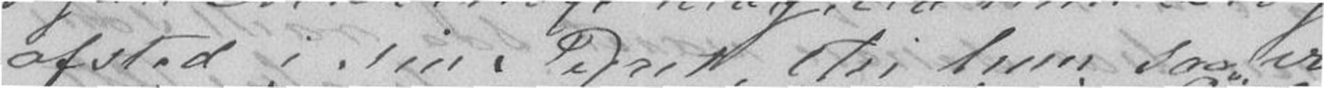afsted i sin Pynt, thi hun saa viBit borte
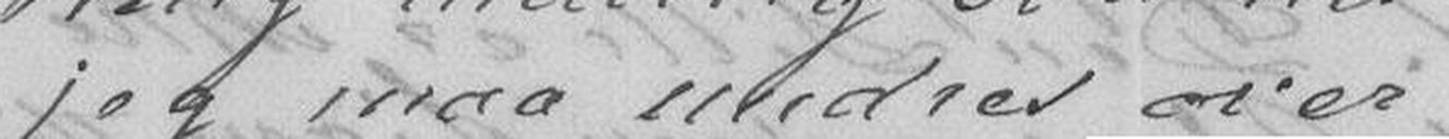jeg maa undres over
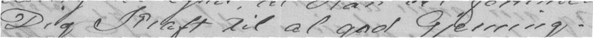Dig Kraft til al god Gjerning.
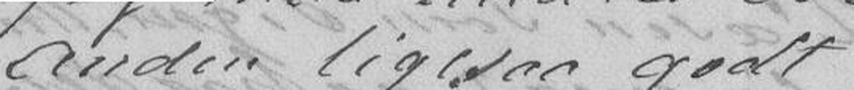anden ligesaa godt
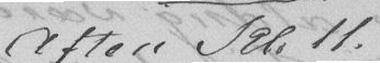Aften Kl.11.
